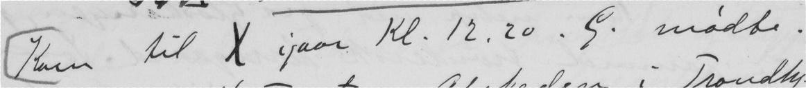Kom til X igaar Kl. 12.20. G. mødte.
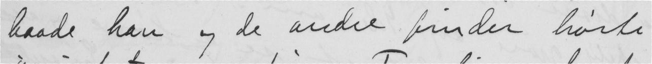baade han og de andre finder hørte
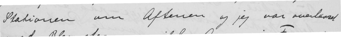Stationen om Aftenen og jeg var overlesset
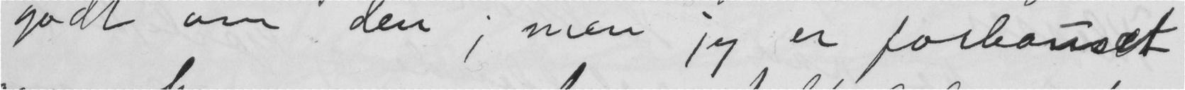godt om den; men jeg er forbauset
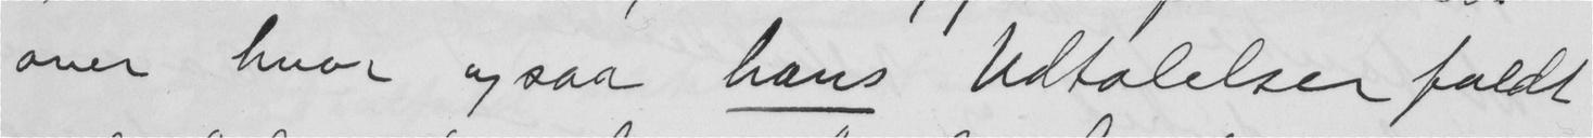over hvor ogsaa hans Udtalelser faldt
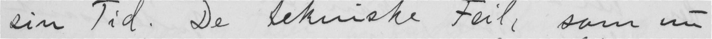sin Tid. De tekniske Feil, som nu
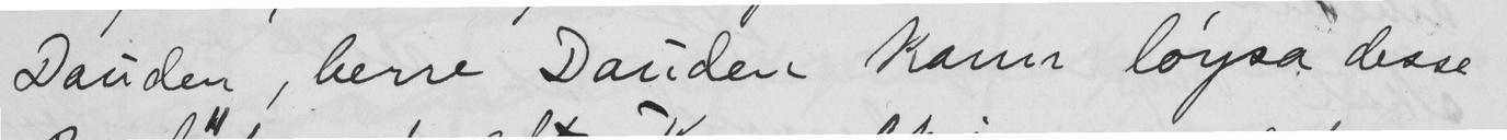Dauden, berre Dauden kann løysa desse
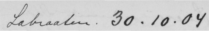Labraaten. 30.10.04
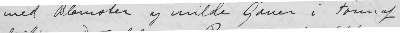med Blomster og milde Gaver i Form af
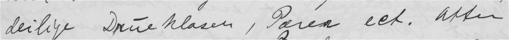deilige Drueklaser, Pærer ect. Atter
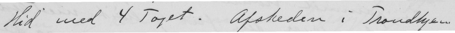Hid med 4 Toget. Afskeden i Trondhjem
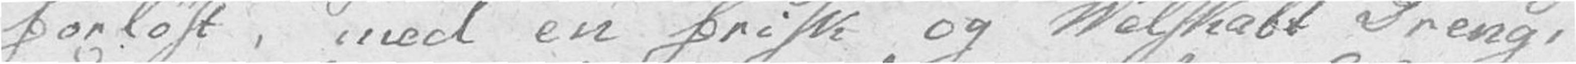forløst, med en frisk og Velskabt Dreng,
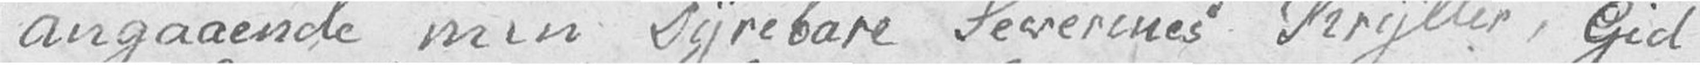angaaende min Dyrebare Severines Kryller, Gid
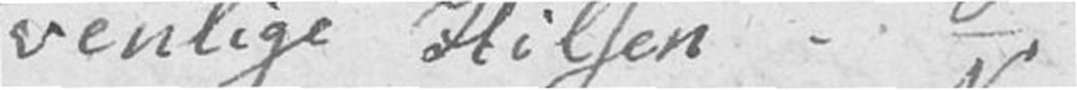venlige Hilsen. –
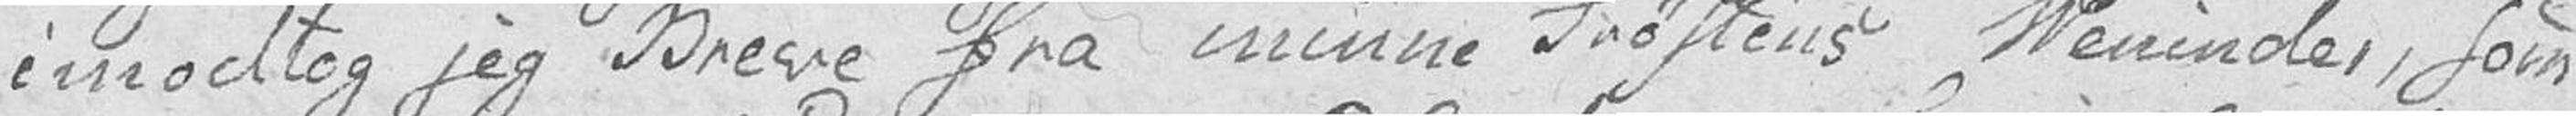imodtog jeg Breve fra minne Trøstens Veninder, som
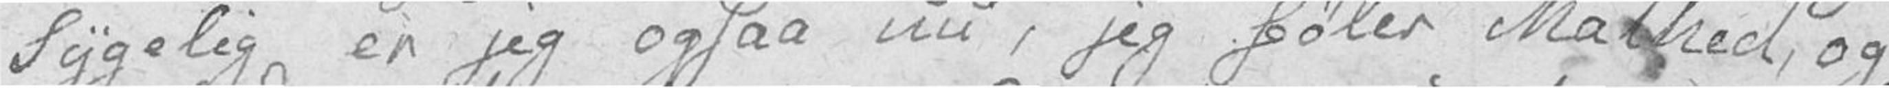Sygelig er jeg ogsaa nu, jeg føler Mathed, og
